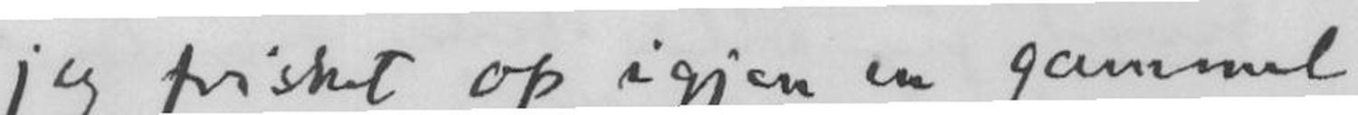jeg frisket op igjen en gammel
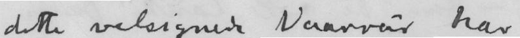dette velsignede Vaarvær har
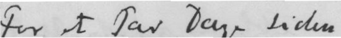For et Par Dage siden
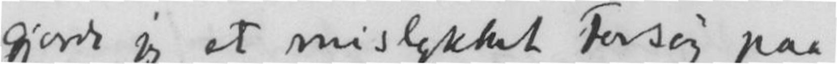gjorde jeg et mislykket Forsøg paa
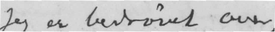Jeg er bedrøvet over
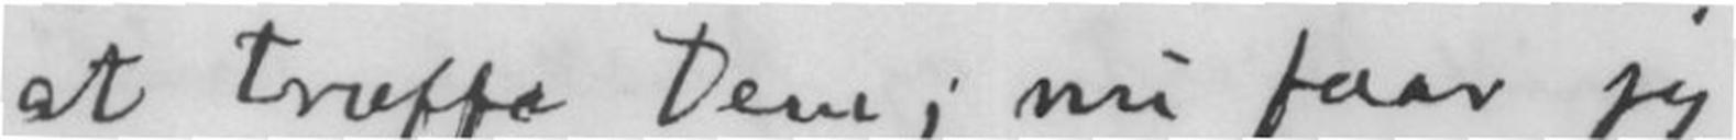at træffe Dem; nu faar jeg
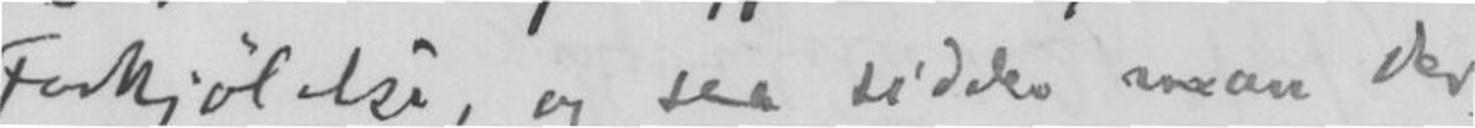Forkjølelse, og saa sidder man der
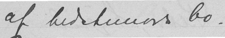af bedstemors bo.
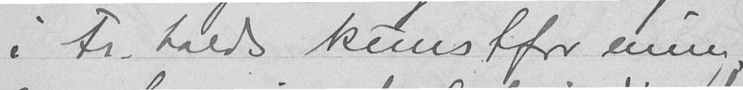i Fr.halds kunstforening
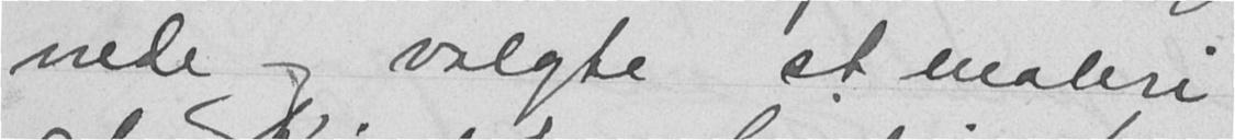nede og valgte et maleri
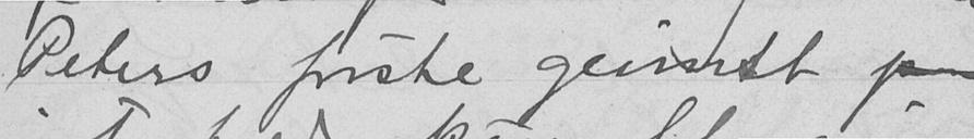Peters første gevinst paa
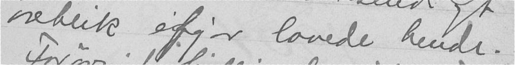øieblik ifjor lovede hende.
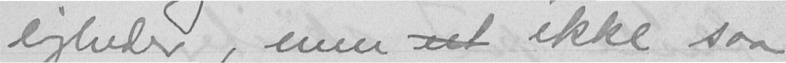ligheder, men eet ikke saa
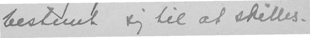bestemt sig til at skilles.
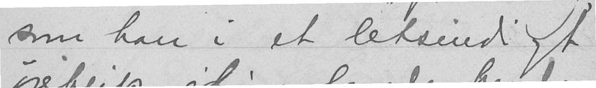som han i et letsindigt
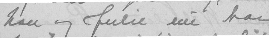han og Julie nu har
